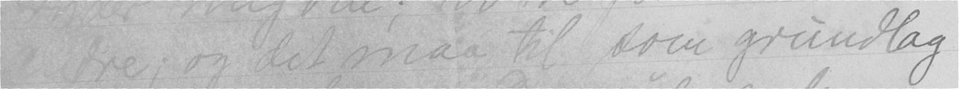andre; og det maa til som grundlag
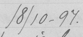18/10-97.
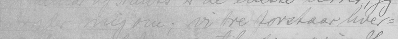der mig om; vi tre forstaar hver-
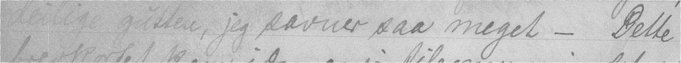deilige gutten, jeg savner saa meget - Dette
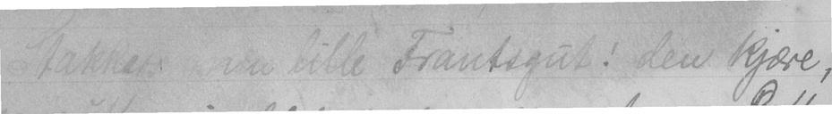Stakkars min lille Frantsgut! den kjære,
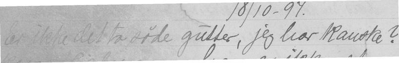Er ikke det to søde gutter, jeg har kanske?
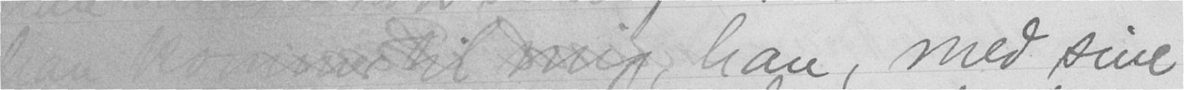han kommer til mig, han, med sine
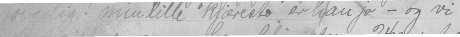følgelig! min lille "kjæreste" er han jo - og vi
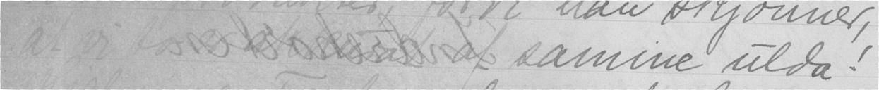at vi to er af samme ulda!
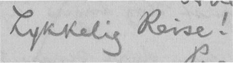Lykkelig Reise!
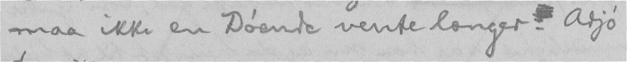maa ikke en Døende vente længer. Adjø
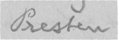Presten
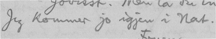Jeg kommer jo igjen i Nat.
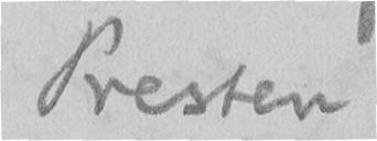Presten
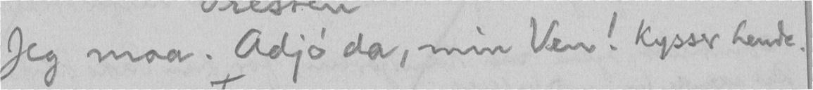Jeg maa. Adjø da, min Ven! kysser hende.
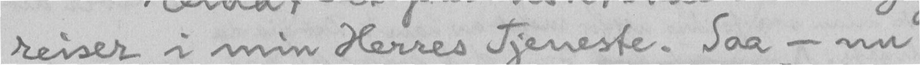reiser i min Herres Tjeneste. Saa - nu
